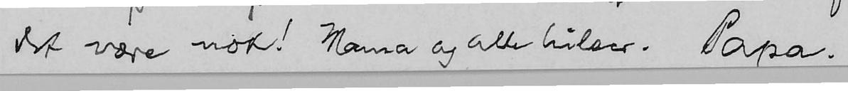det være nok! Mama og alle hilser. Papa.
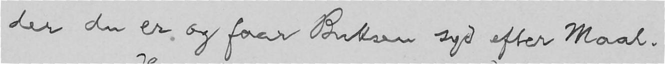der du er og faar Buksen syd efter Maal.
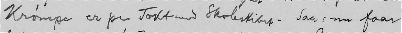Krømpe er paa Tokt med Skoleskibet. Saa, nu faar
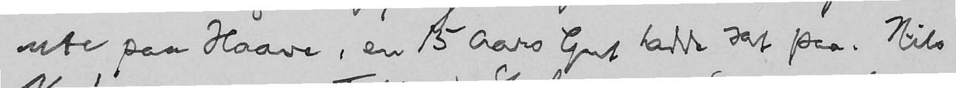ute paa Haave, en 15 Aars Gut hadde sat paa. Nils
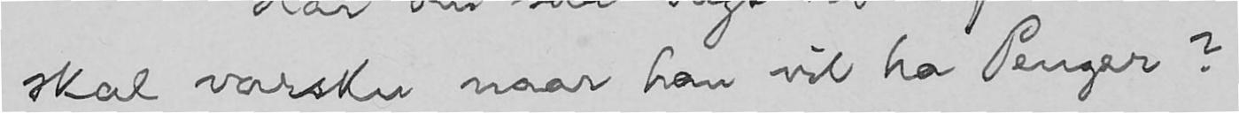skal varsku naar han vil ha Penger?
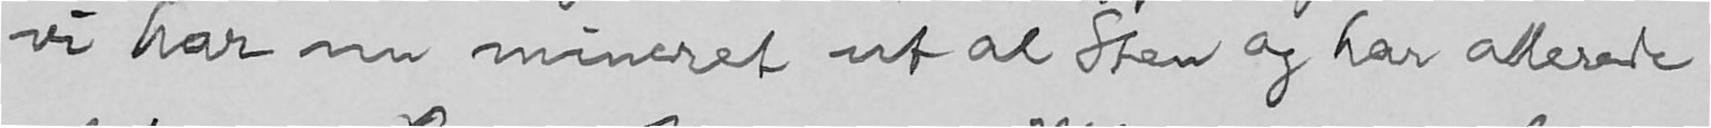vi har nu mineret ut al Sten og har allerede
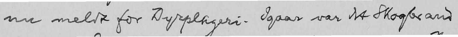nu meldt for Dyrplageri. Igaar var det Skogbrand
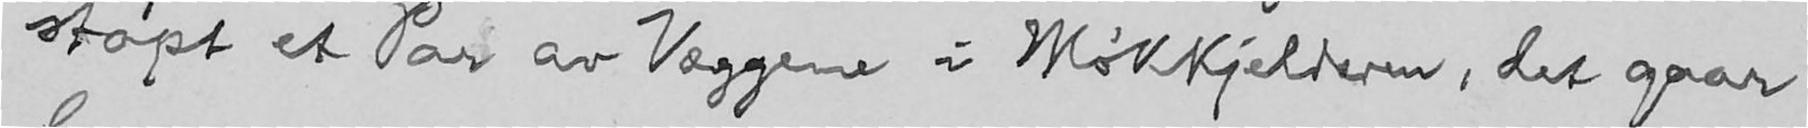støpt et Par av Væggene i Møkkjelderen, det gaar
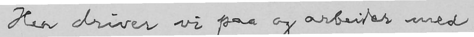Her driver vi paa og arbeider med
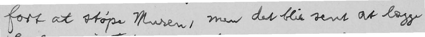fort at støpe Muren, men det blir sent at lægge
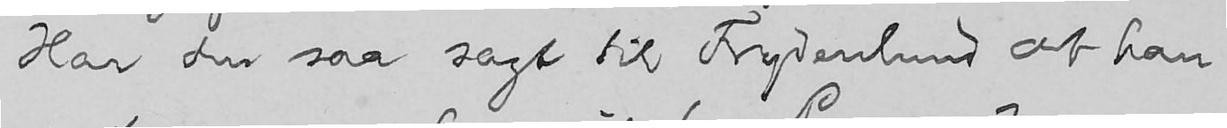Har du saa sagt til Frydenlund at han
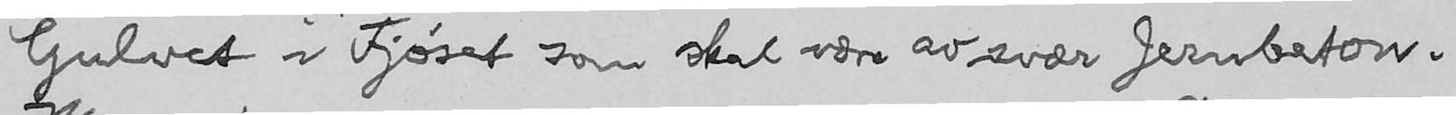Gulvet i Fjøset som skal være av svær Jernbeton.
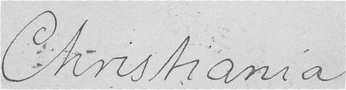Christiania
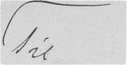Til
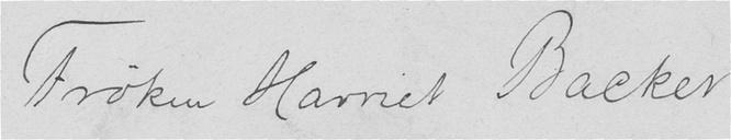Frøken Harriet Backer
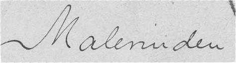Malerinden
